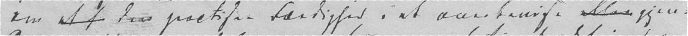om den practiske Færdighed i at overbevise gjen-
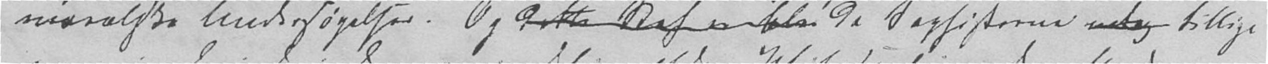moralske Undersøgelser. Og da Sophisterne tillige
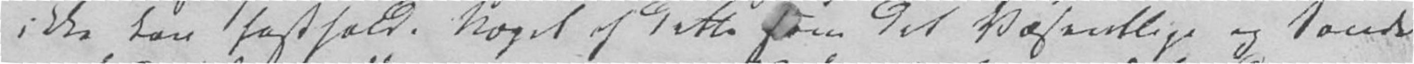ikke kan fastholde Noget af dette som det Væsentlige og Sande
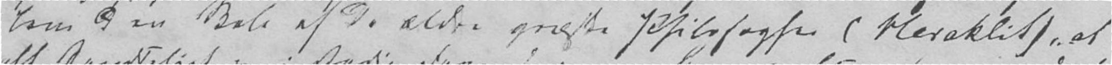lom en Skole af de ældre græske Philosopher (Heraklit), at
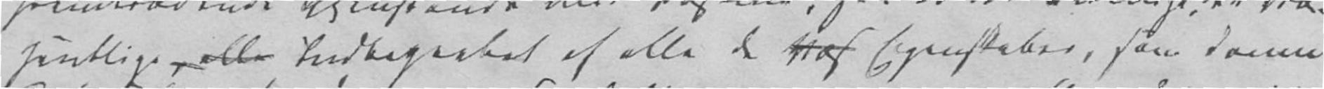sentlige Indbegrebet af alle de Egenskaber, som danne
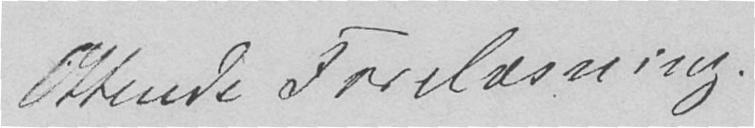Ottende Forelæsning.
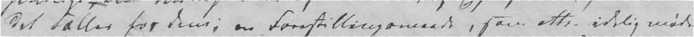det Fælles for dem; en Forestillingsmaade, som atter idelig møder
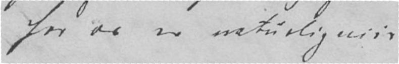for os er naturligviis
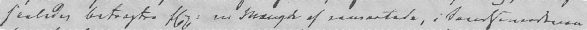saaledes betragter fEx: en Mængde af eensartede, i Sandseverdenen
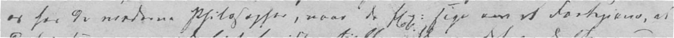os hos de moderne Philosopher, naar de fEx: sige om et Fortegions, at
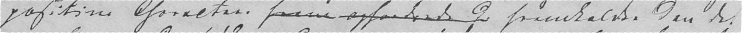positive Character fremkaldte den de
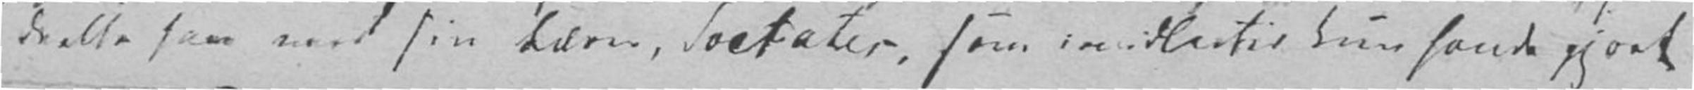deelte han med sin Lærer, Socrates, som imidlertid kun havde gjort
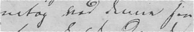netop ved denne sin
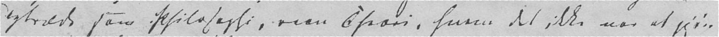optræde som Philosophi, men Theori, hvem det ikke var at gjøre
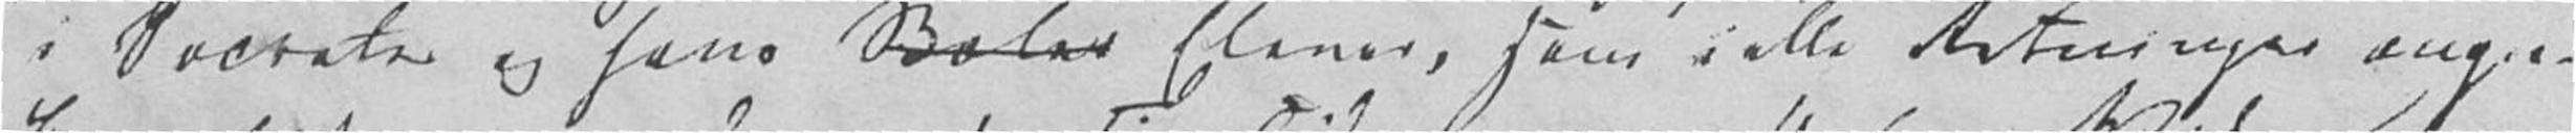i Socrates og hans Elever, som i alle Retninger angre-
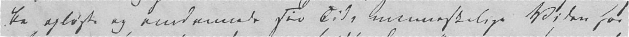be opløste og vindennede sin Tids menneskelige Viden for
